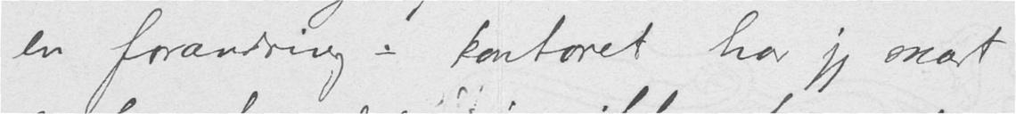en forandring - kontoret har jeg snart
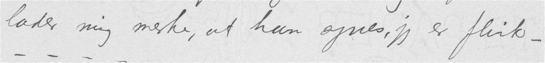lader mig merke, at han synes, jeg er flink -
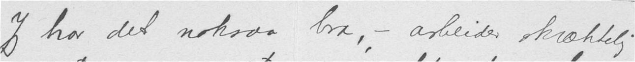Jeg har del noksaa bra, - arbeider skrækkelig
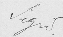Sigrid.
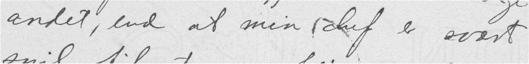andet, end at min chef er svært
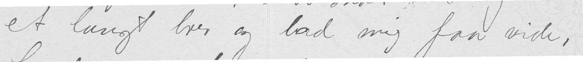et langt brev og lad mig faa vide,
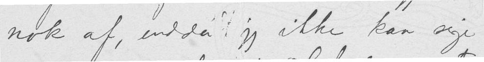nok af, endda jeg ikke kan sige
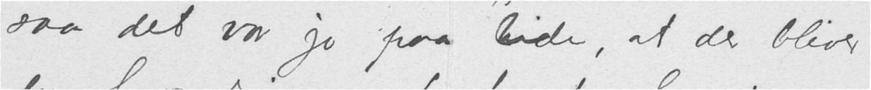saa det var jo paa tide, at der bliver
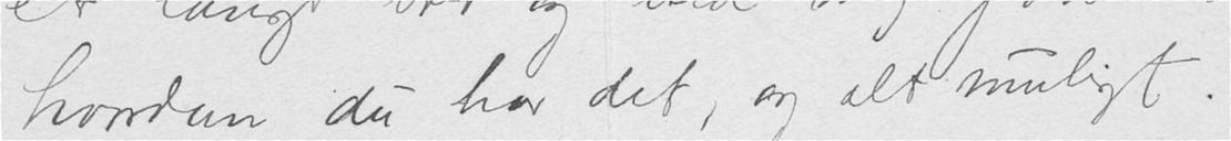hvordan du har det, og alt muligt.
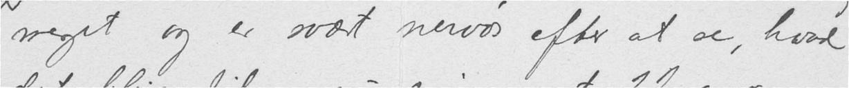meget og er svært nervøs efter at se, hvad
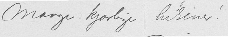Mange kjærlige hilsener!
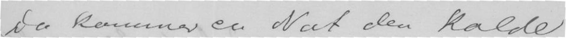5 Da kommer en Nat den kalde
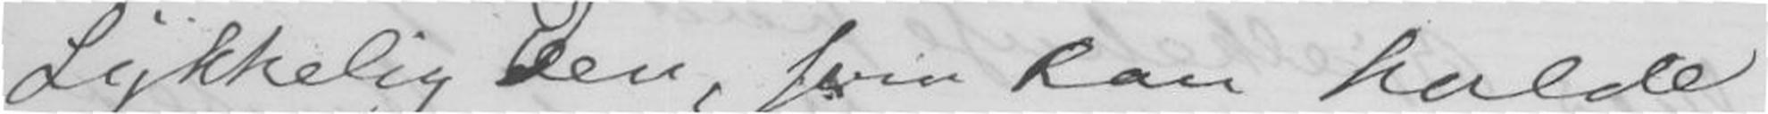Lykkelig den, som kan kalde
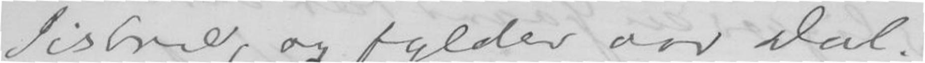Iisbre, og fylder vor Dal.
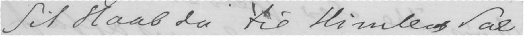Sit Haab da til Himlens Sal!
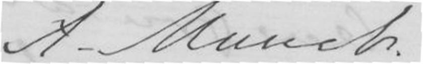A. Munch.
